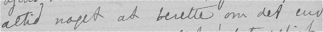altid noget at berette, om det end
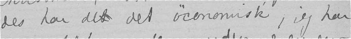des har det det øconomisk, jeg har
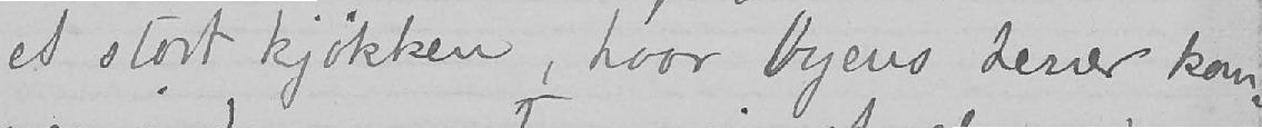et stort kjøkken, hvor byens herrer kom-
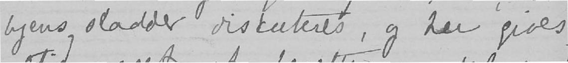byens sladder diskuteres, og her gives
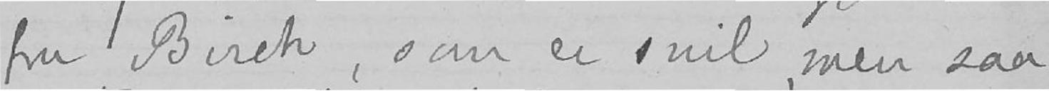fru Birch, som er snil, men saa
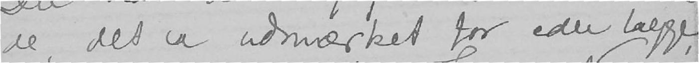de, det er udmærket for eder begge,
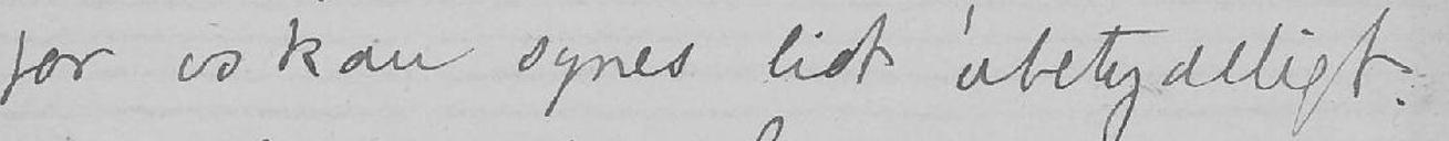for os kan synes lidt ubetydeligt.
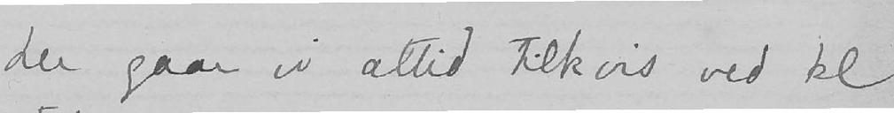der gaar vi altid tilkøis ved kl
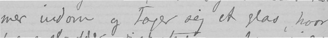mer indom og tager sig et glas, hvor
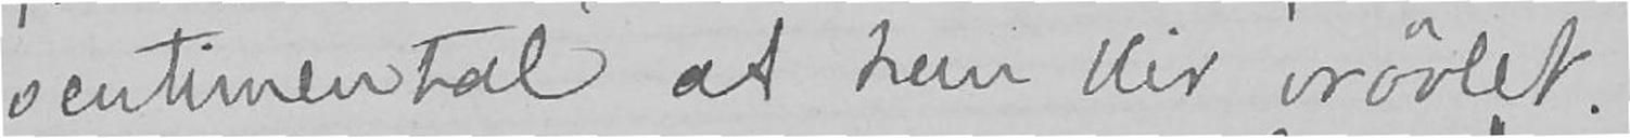sentimental at hun blir vrøvlet.
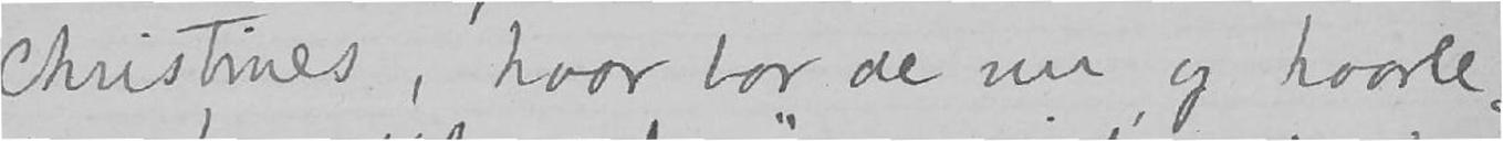Christines, hvor bor de nu, og hvorle-
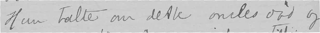Hun talte om dette oncles død og
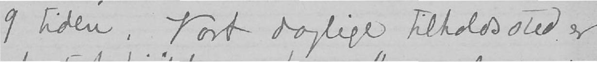9 tiden. Vort daglige tilholdssted er
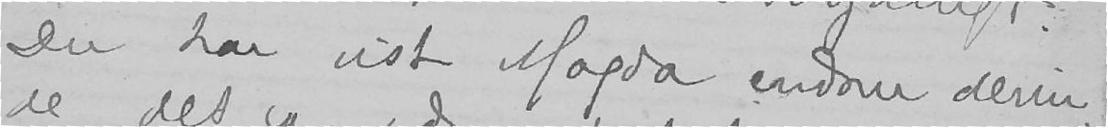Du har vist Magda endnu derin-
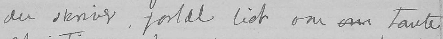du skriver, fortæl lidt om om tante
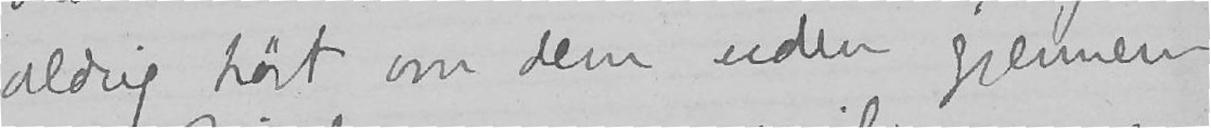aldrig hørt om dem uden gjennem
